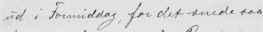ud i Formiddag, for det snede saa
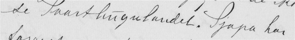se Svarthuguvatnet, Sjapa har
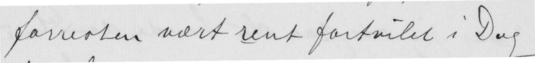forresten vært rent fortvilet i Dag
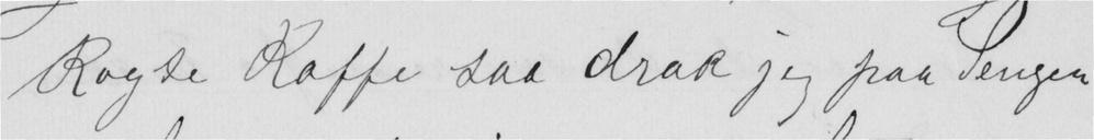Kogte Kaffe saa drak jeg paa Sengen
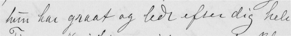hun har graat og ledt efter dig hele
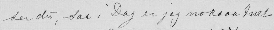ser du, saa i Dag er jeg noksaa træt
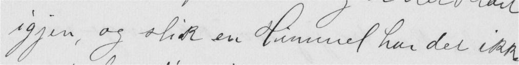igjen, og slik en Himmel har det ikke
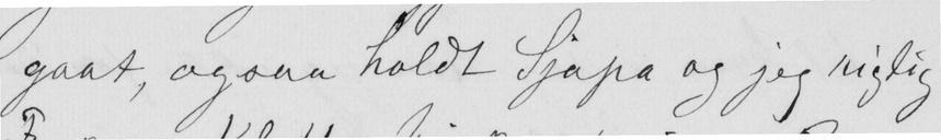gaat, ogsaa holdt Sjapa og jeg rigtig
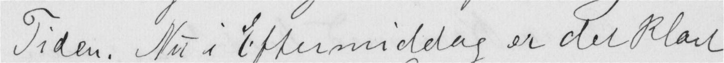Tiden. Nu i Eftermiddag er det Klart
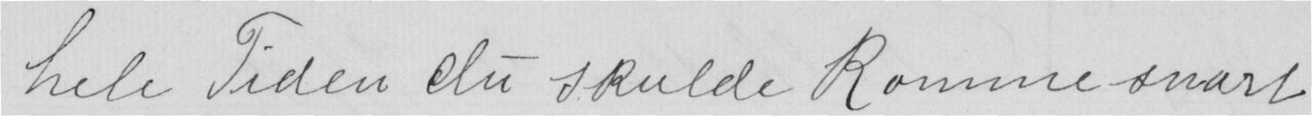hele Tiden du skulde Komme snart
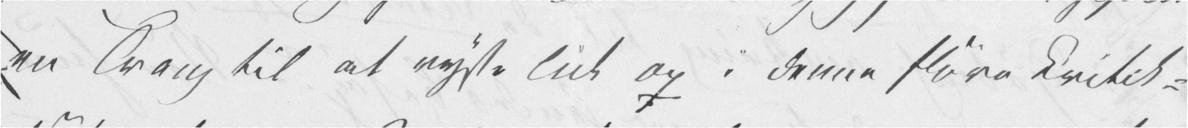en Trang til at ryste lidt op i denne sløve Kritik-
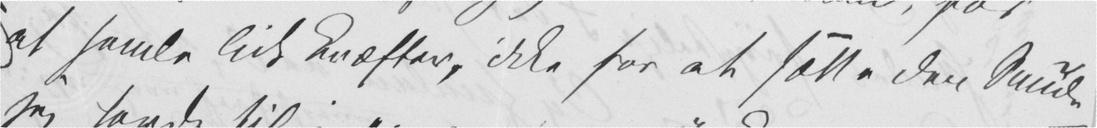at samle lidt Kræfter, ikke for at sætte den Smule
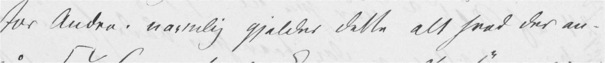for Andre. navnlig gjelder dette alt hvad der an-
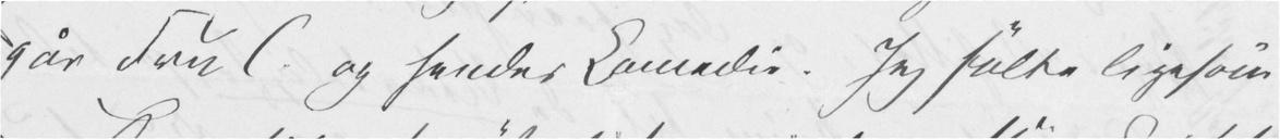går Fru C. og hendes Komedie. Jeg følte ligesom
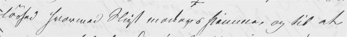løshed hvormed Sligt modtages hjemme, og til at
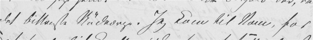det bittreste Nødværge. Jeg kom til Rom, for
